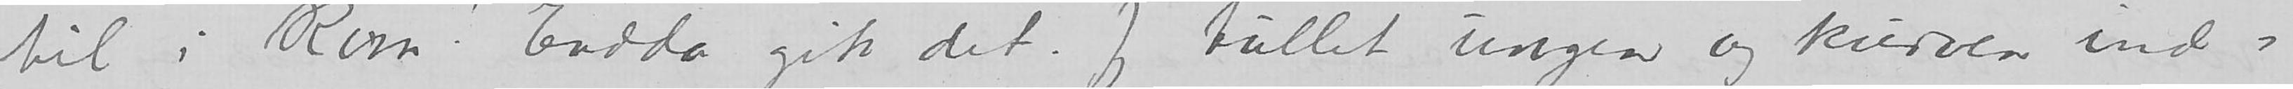til i Rom! Endda gik det. Jeg tullet ungen og kurven ind i
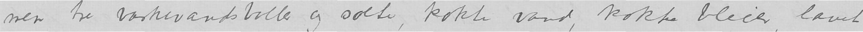men tre vaskevands boller og sølte, kokte vand, kokte bleier, lavet
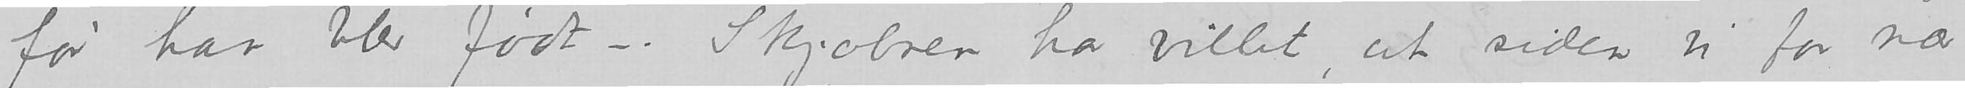før han blev født –. Skjæbnen har villet, at siden vi for nær
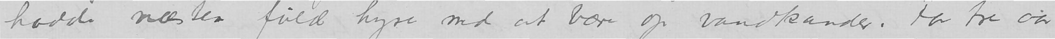hadde næsten fuld hyre med at bære op vandkander. For tre aar
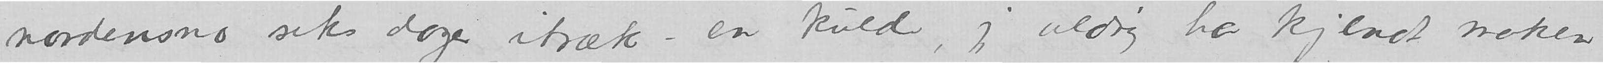nordensno seks dager itræk – en kulde, jeg aldrig har kjendt maken
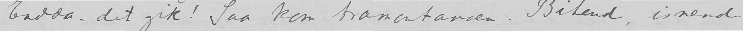– Endda – det gik! Saa kom tramontanaen. Bitende, isnende
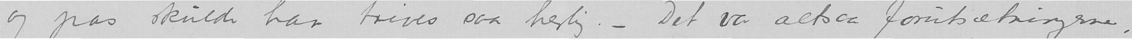og pas skulde han trives saa herlig. – Det var altsaa forutsætningerne,
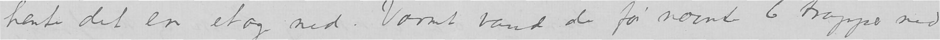hænte det en etage ned. Varmt vand de før nævnte 6 trapper ned.
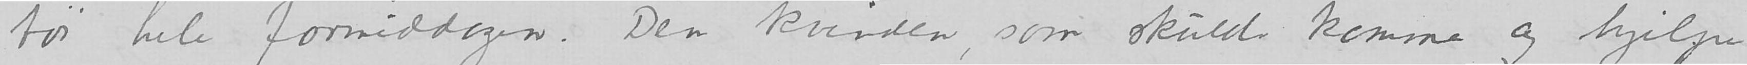tøi hele formiddagen. Den kvinden, som skulde komme og hjelpe
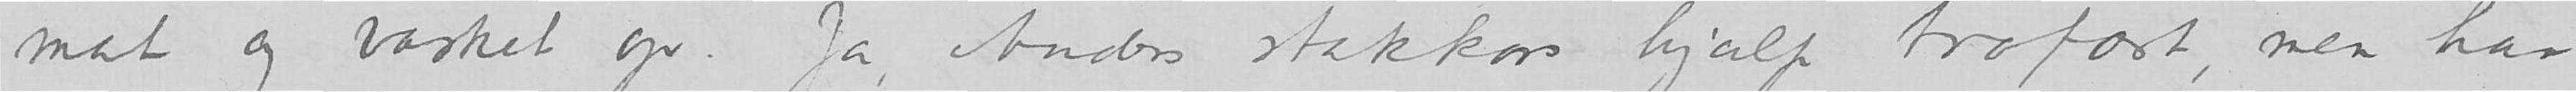mat og vasket op. Ja, Anders stakkars hjalp trofast, men han
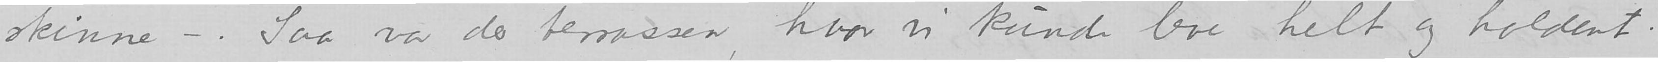skinne –. Saa var der terrassen, hvor vi kunde leve helt og holdent.
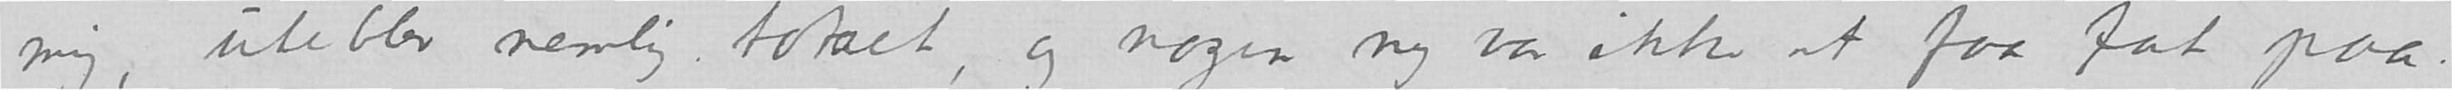mig, uteblev nemlig totalt, og nogen ny var ikke at faa fat paa.
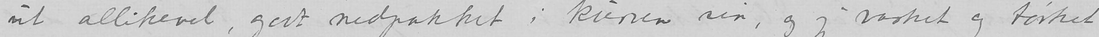ut allikevel, godt nedpakket i kurven sin, og jeg vasket og tørket
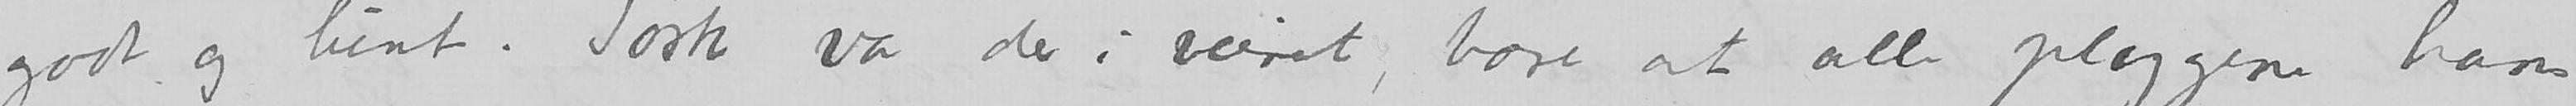godt og lunt. Tørk var der i veiret, bare at alle plaggerne hans
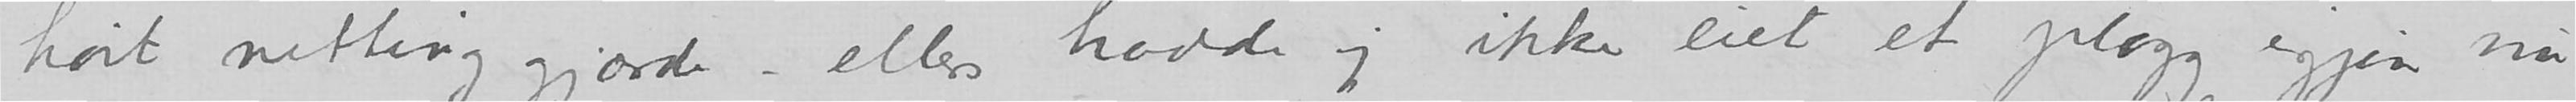høit netting gjærde – ellers hadde jeg ikke eiet et plagg igjen nu.
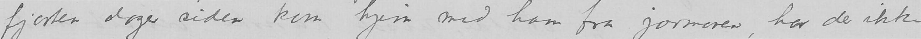fjorten dager siden kom hjem med ham fra jormoren, har der ikke
